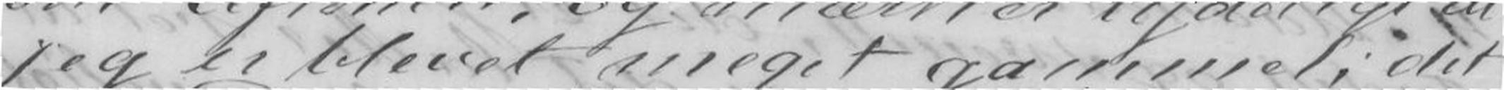jeg er blevet meget gammel; det
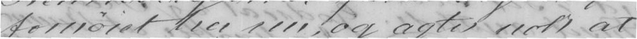fornøiet her nu, og agter nok at
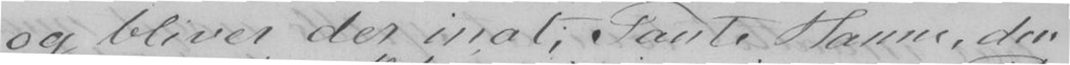og bliver der inat, Tante Hanne, den
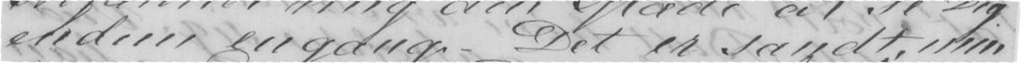endnu engang. Det er sandt, min
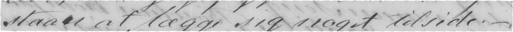staaer at lægge sig noget tilside. -
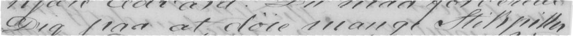Dig paa at døie mange Stikpiller
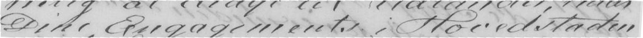Dine Engagements i Hovedstaden
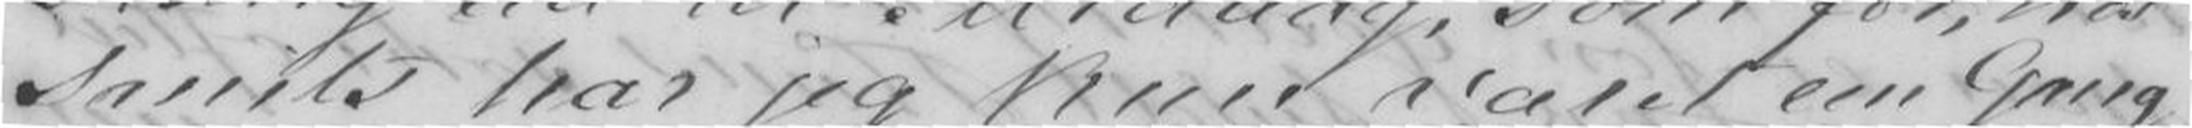Smits har jeg kun været en Gang
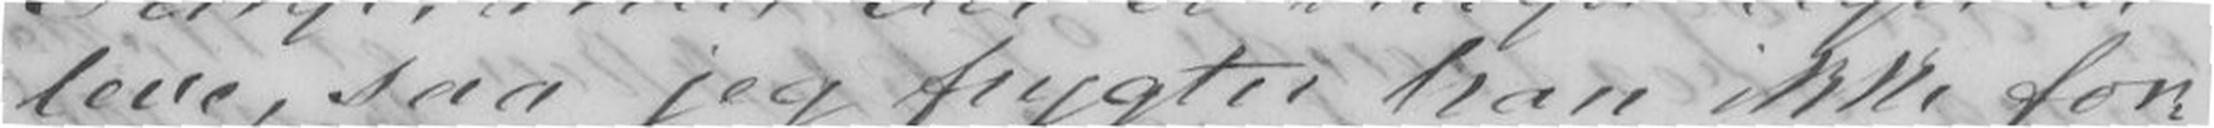leve, saa jeg frygter han ikke for-
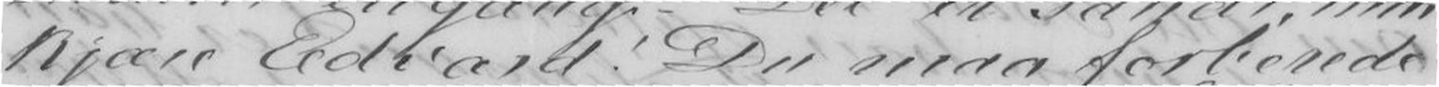kjære Edvard! Du maa forberede
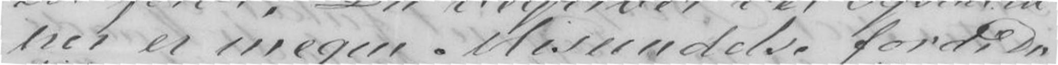her er megen Mesundelse fordi at Du
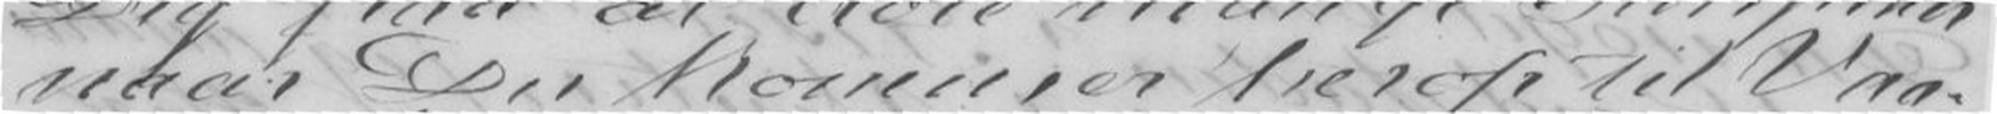naar du kommer herop til Vaa-
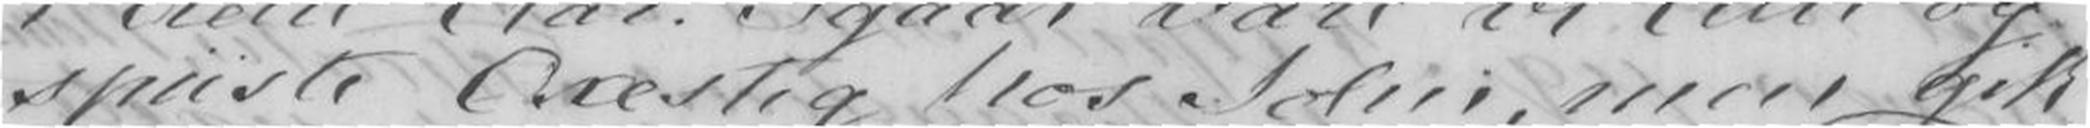spiiste Oxesteg hos John, men gik
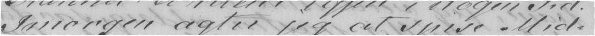Imorgen agter jeg at spise Mid-
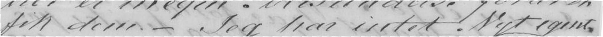fik dem. - Jeg har intet Nyt egent-
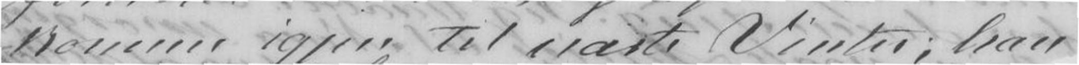komme igjen til næste Vinter; han
